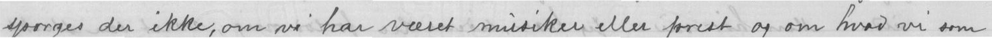spørges der ikke, om vi har været musiker eller prest og om hvad vil som
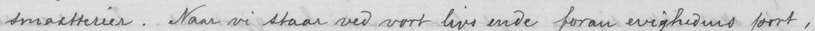smaaterier. Naar vi staar ved vort livs ende foran evighedens port,
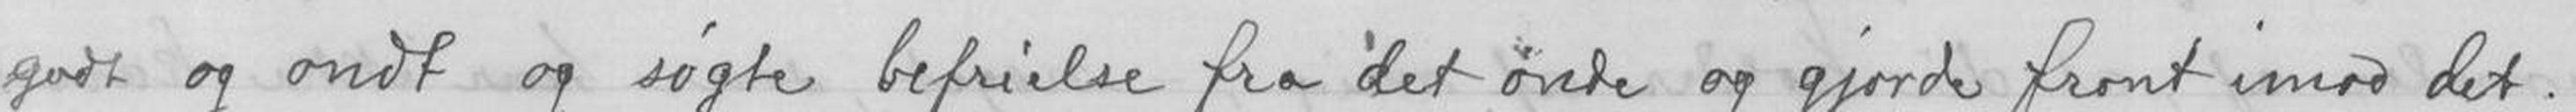godt og ondt og søgte befrielse fra det onde og gjorde front imod det.
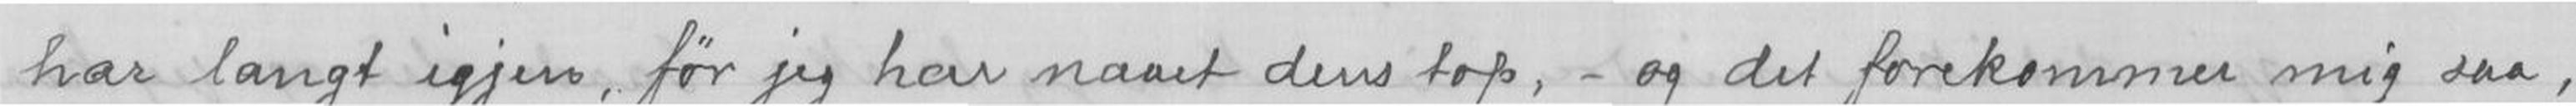har langt igjen, før jeg har naaet dens top, - og det forekommer mig saa,
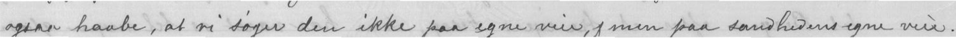ogsaa haabe, at vi søger den ikke paa egne veier, men paa sandhedens egne veie.
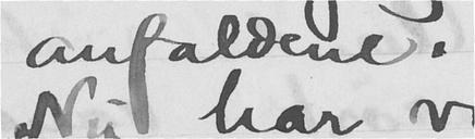anfaldene.
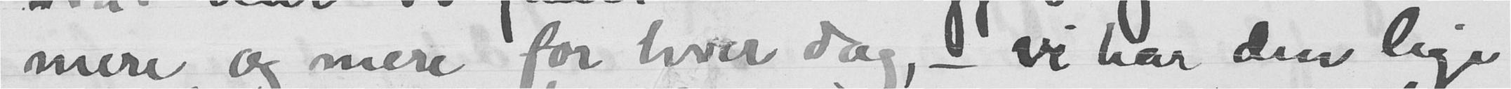mere og mere for hver dag, - vi har den lige
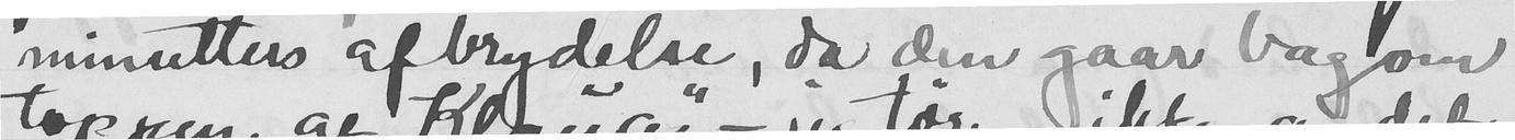minutters afbrydelse, da den gaare bagom
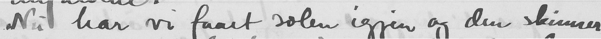Nu har vi faaet solen igjen og den skinner
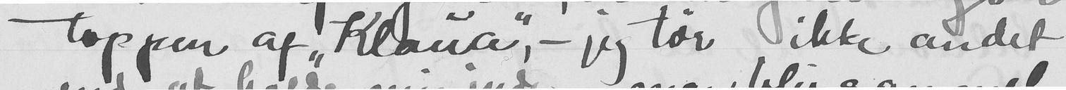toppen af "Klaua", - jeg tør ikke andet
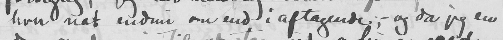hver nat endnu om end i aftagende; - og da jeg en
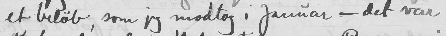et beløb, som jeg modtog i januar, - det var
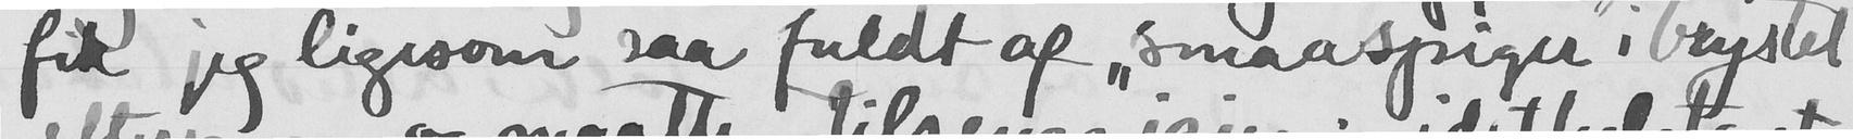fik jeg ligesom saa fuldt af "smaaspiger" i brystet
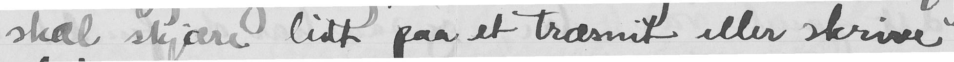skal skjære lidt paa et træsnit eller skrive
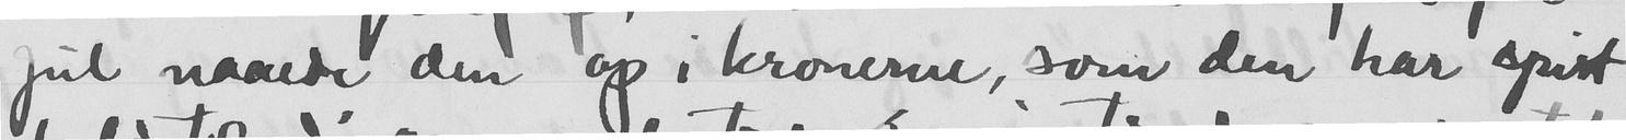jul naaede den op i kronerne, som den har spist
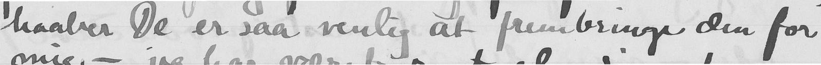haaber De er saa venlig at frembringe den for
